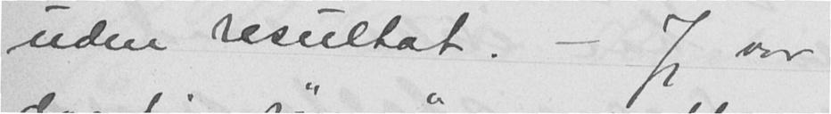uden resultat. - Jeg var
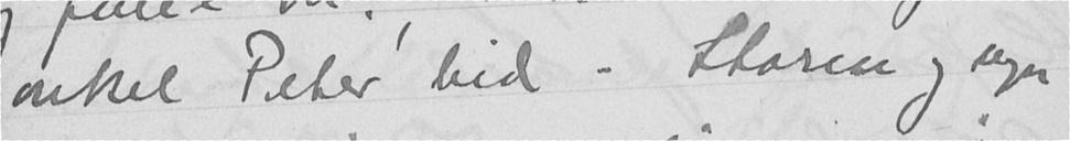onkel Peter hid. Storm og regn
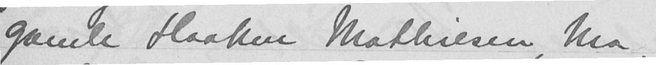gamle Haaken Mathiesen, Ma,
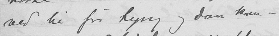ved hi før Lyng og Don kom -
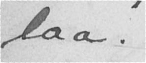laa.
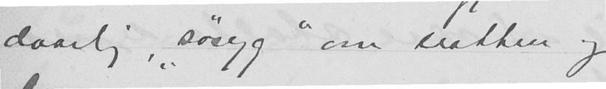daarlig, "søsyg" om natten og
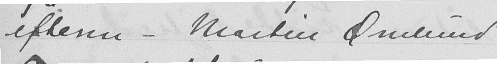efterm. Martin Ørnelund
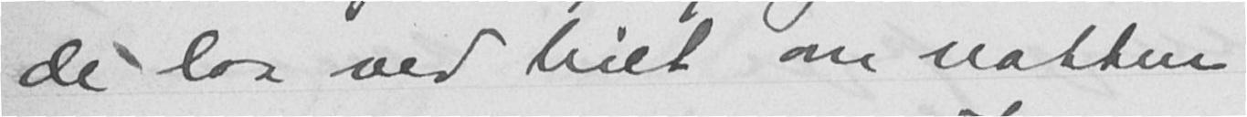de laa ved hiet om natten
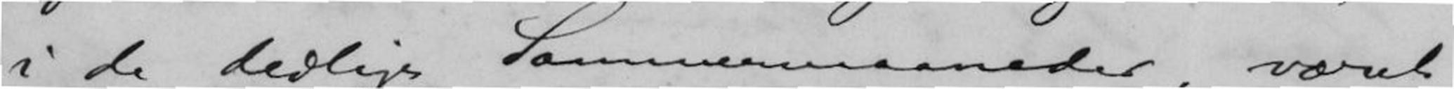i de deilige Sommermaaneder, været
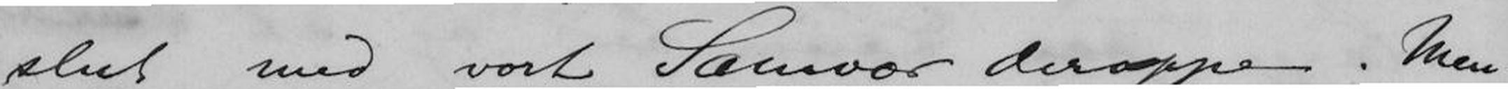slut med vort Samvær deroppe. Men
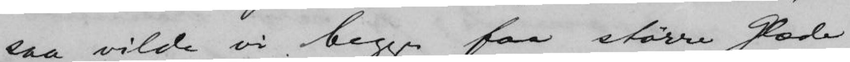saa vilde vi begge faa større Glæde
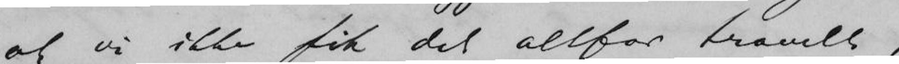at vi ikke fik det altfor travelt,
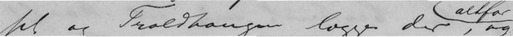set og Troldhaugen ligge der, og
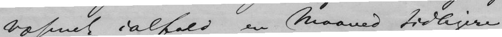væsenet ialtfald en Maaned tidligere
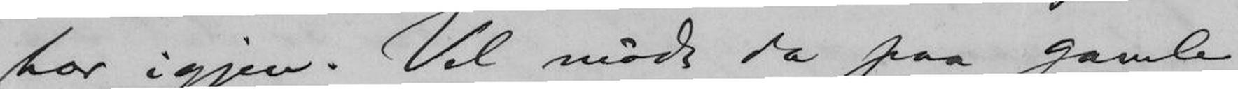har igjen. Vel mødt da paa gamle
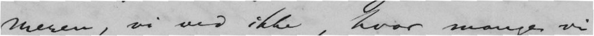meren, vi ved ikke, hvor mange vi
